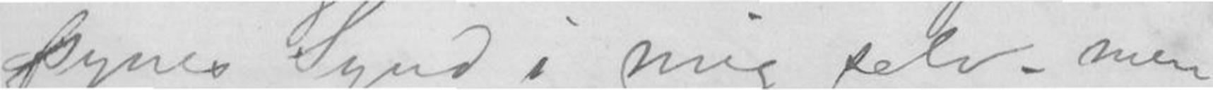synes Synd i mig selv _ men
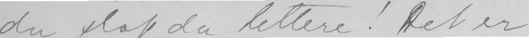du slap da lettere! Det er
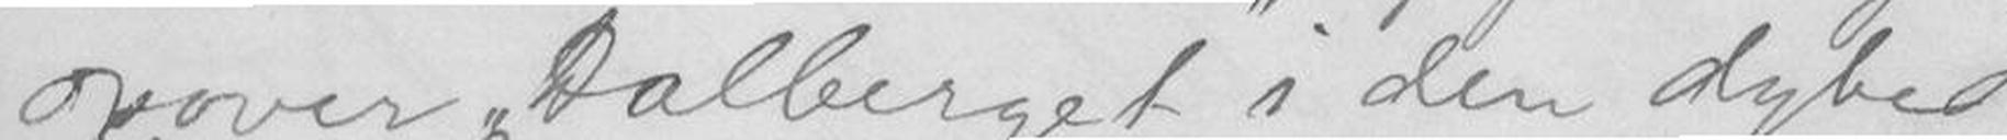opover Dalberget i den dybe
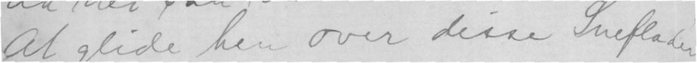At glide hen over disse Sneflader
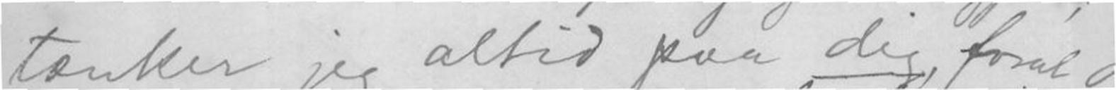tænker jeg altid paa dig, forat
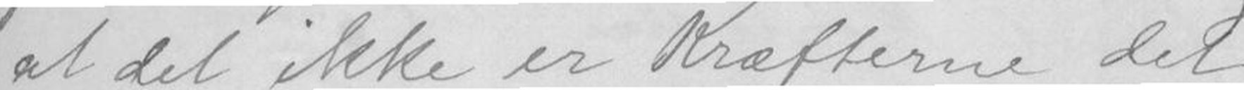at det ikke er Kræfterne det
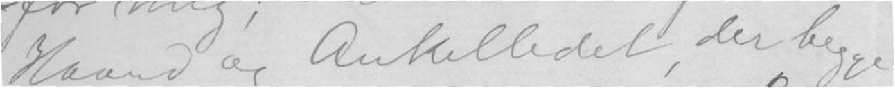Haand og Ankelledet, der begge
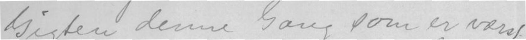Gigten denne Gang som er værst
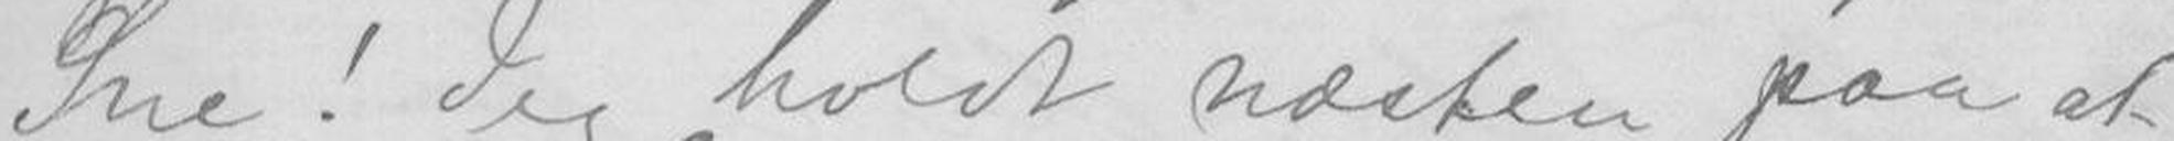Sne! Jeg holdt næsten paa at
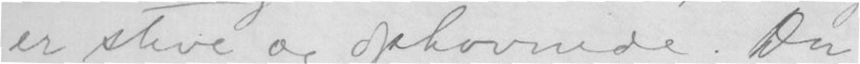er stive og ophovnede. Du
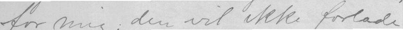for mig; den vil ikke forlade
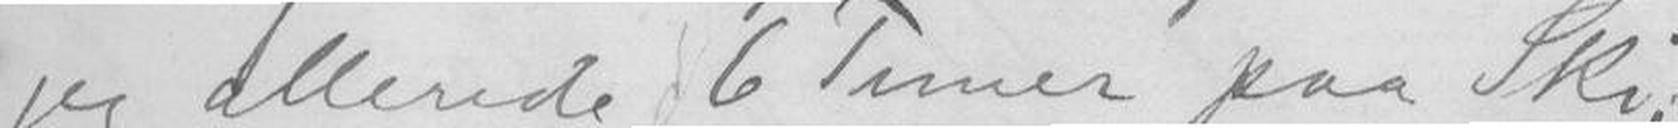jeg allerede 6 Timer paa Ski;
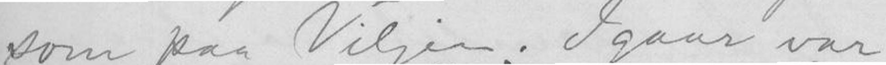som paa Viljen. Igaar var
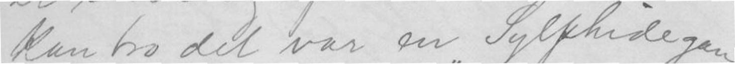kan tro det var en Sylphidegang
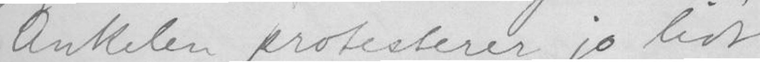Ankelen protesterer jo lidt
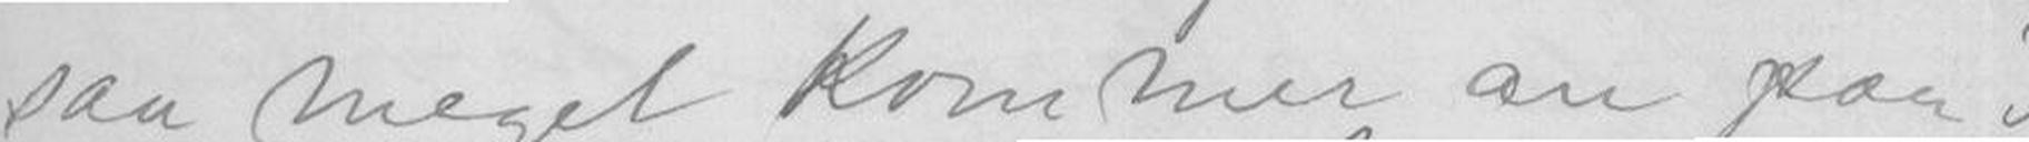saa meget kommer an paa
